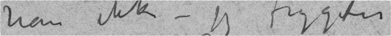han ikke – jeg frygter
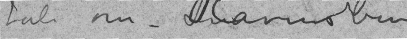tale om – LuRavensberg
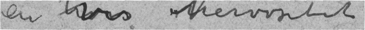en hvis Nervøsitet
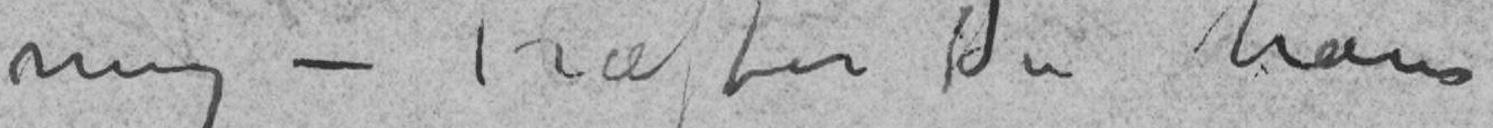mig – Træffer Du ham
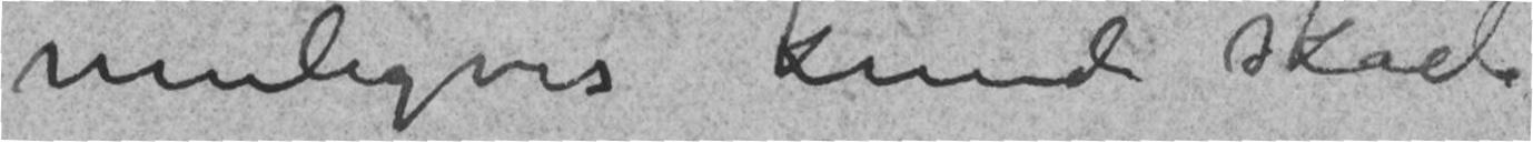muligvis kunde skade
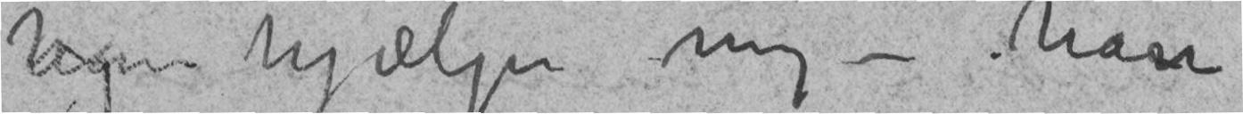og hjælpe mig – han
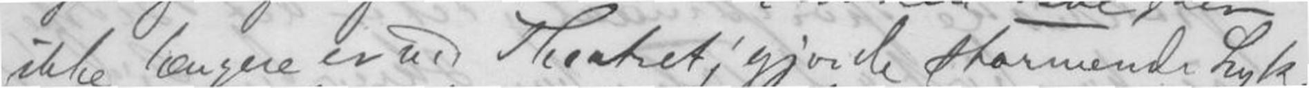ikke længere er ved Theatret; gjorde stormende Lyk-
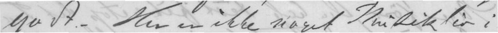godt. Her er ikke noget Musikliv i
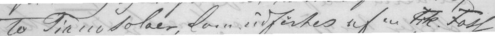to Pianosoloer, som udførtes af en Frk. Foss
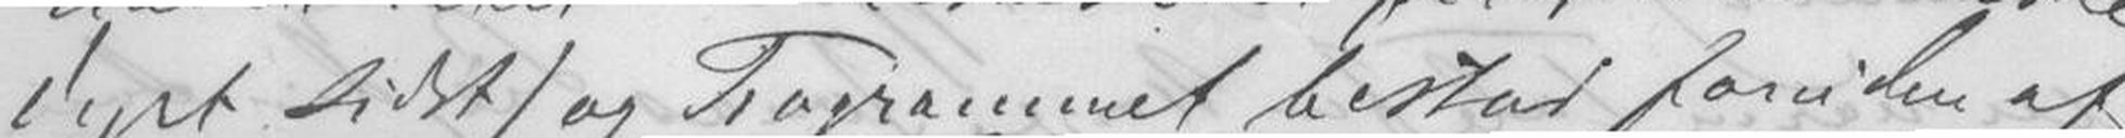dyrt sidst) og Programmet bestod foruden af
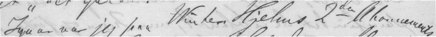Igaar var jeg paa Winter Hjelms 2den Abonnements
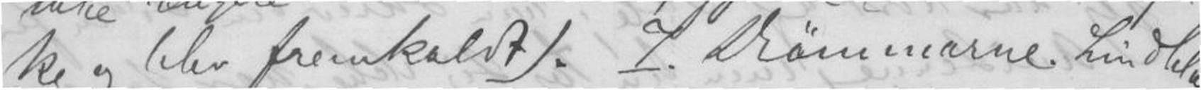ke og blev fremkaldt). 7. Drømmarne. Lindblad.
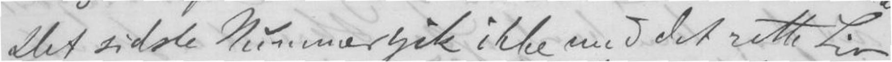Det sidste Nummer gik ikke med det rette Liv.
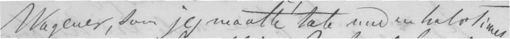Wagner, som jeg maatte tale med en halv Times
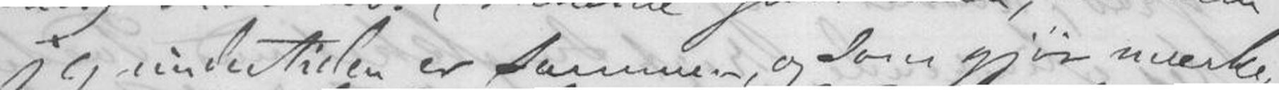jeg undertiden er sammen, og som gjør mærke-
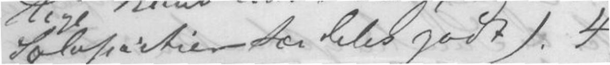Solopartier særdeles godt). 4
